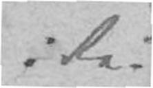i Re-
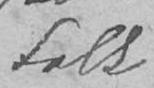Folk
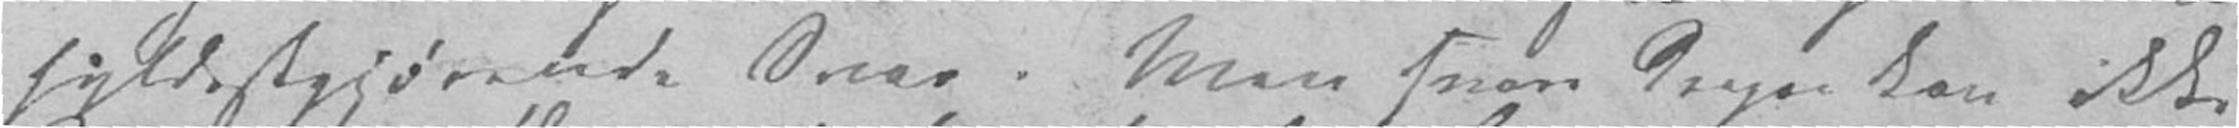fyldestgjørende Svar. Men svare derpaa kan ikke
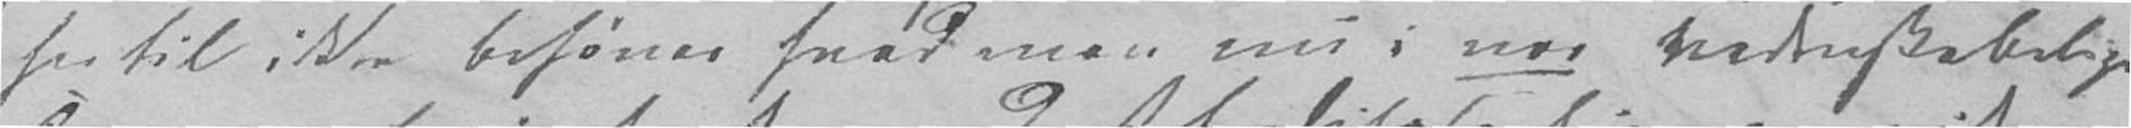hertil ikke behøves hvad man nu i vor videnskabelige
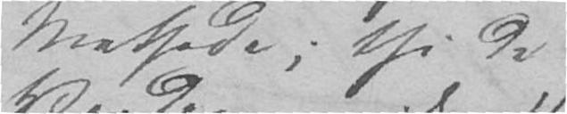Methode; thi de
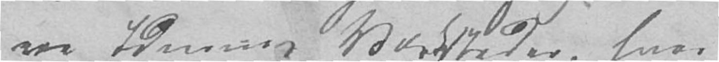ere Ideenes Veksteder, hvor
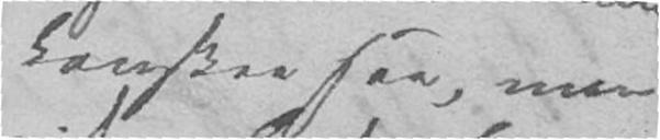kanskee saa, men
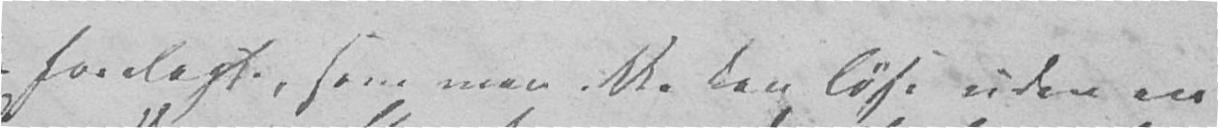forelagt, som man ikke kan løse uden en
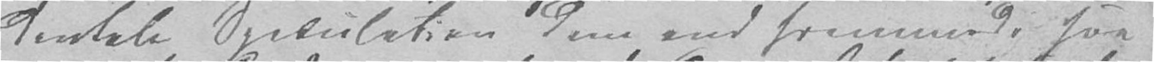dentale Speculation dem end fremmed, saa
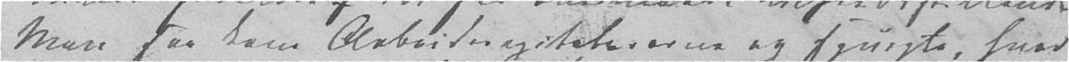Men saa kom Arbeideragitatorerne og spurgte, hvad
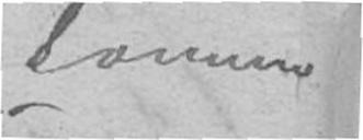kommer
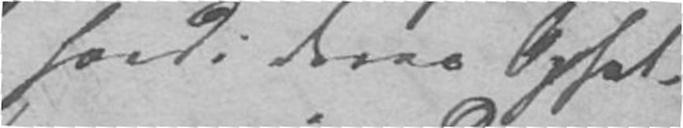fordi deres Opfat-
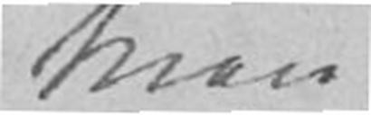Man
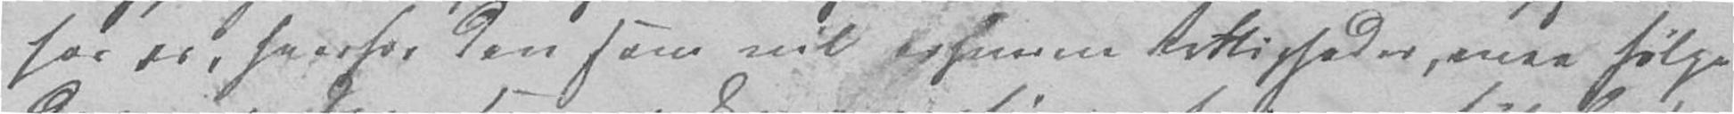hos os, hvorfor den som vil erhverve Rettigheder, maa følge
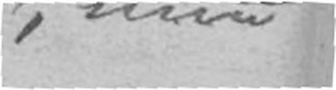, men
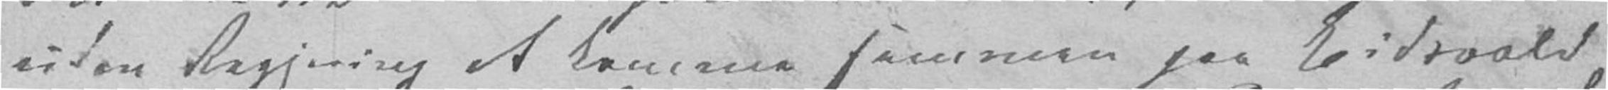uden Regjering at komme sammen paa Eidsvold
